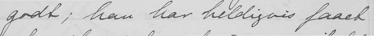godt; han har heldigvis faaet
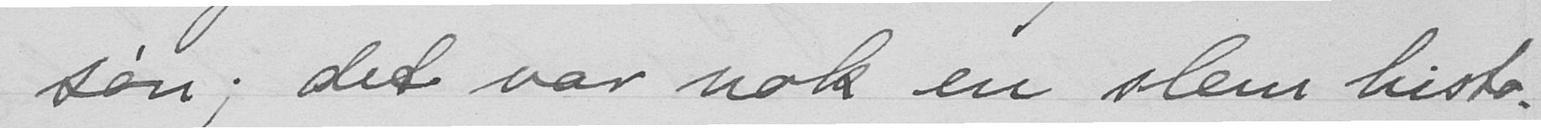søn; det var nok en slem histo-
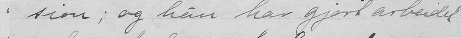sion; og hun har gjort arbeidet
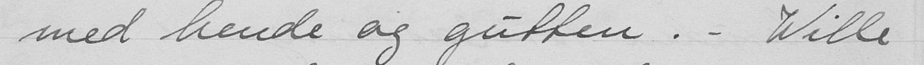med hende og gutten. - Wille
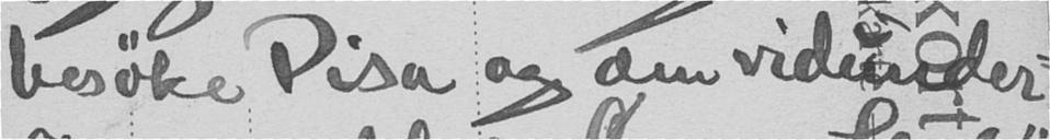besøke Pisa, og den vidunder-
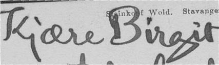Kjære Birgit
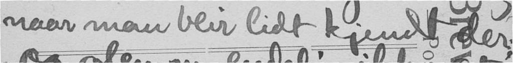naar man blir lidt kjendt der.
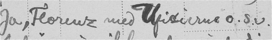Ja, Florenz med Uficierne o.s.v.
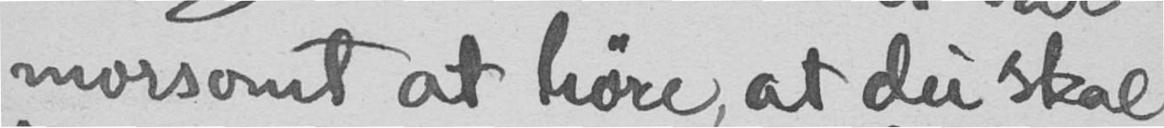morsomt at høre, at du skal
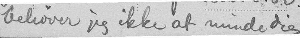behøver jeg ikke at minde dig
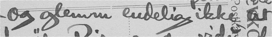- og glemm endelig ikke at
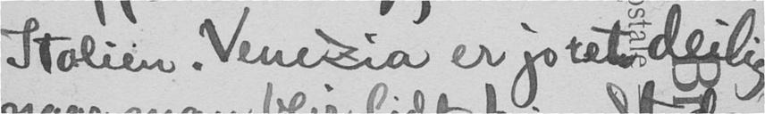Italien. Venezia er jo ret rdeilig
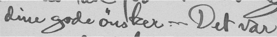dine gode ønsker. - Det var
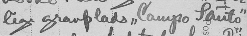lige gravplads "Campo Santo"
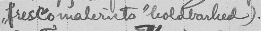"frescomaleriets holdbarhed).
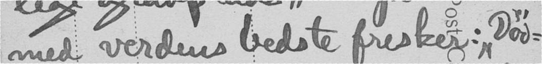med verdens bedste fresker: "Død-
